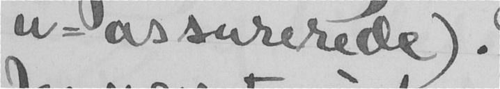u-assurerede).
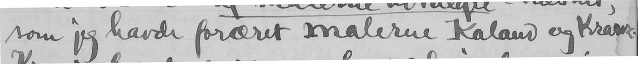som jeg havde foræret svaalerne Kaland og Kranz.
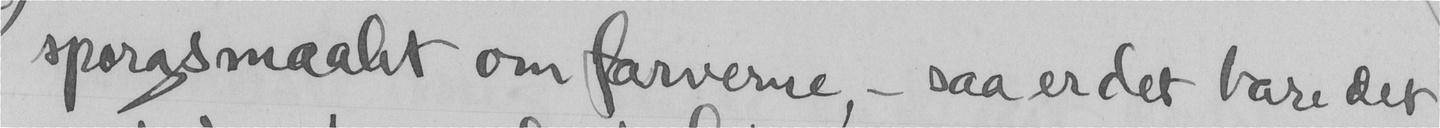sporgsmaalet om farverne - saa er det bare det
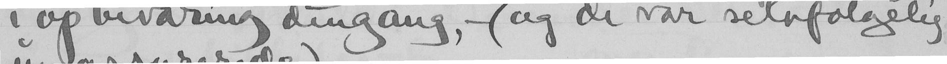i opbevaring dengang, - (og de var selvfølgelig
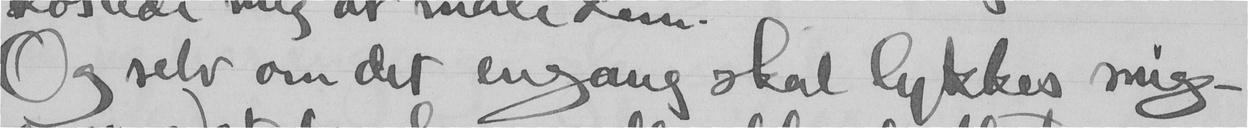Og selv om det engang skal lykkes mig -
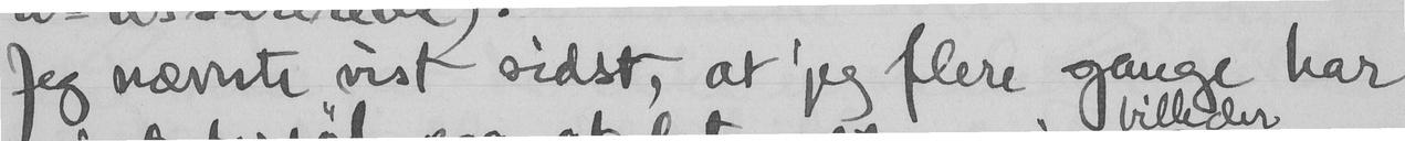Jeg nævnte vist sidst, at jeg flere gange har
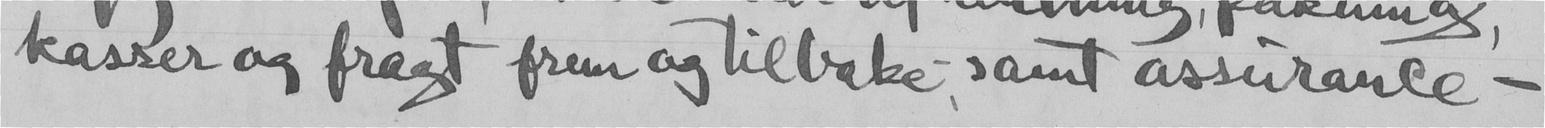kasser og fragt frem og tilbake samt assurance -
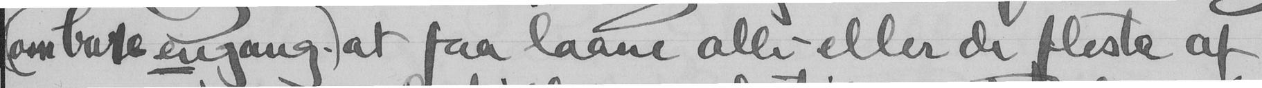(om bare engang) at faa laane alle eller de fleste af
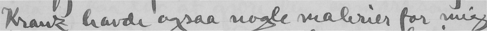Kranz havde ogsaa nogle malerier for mig
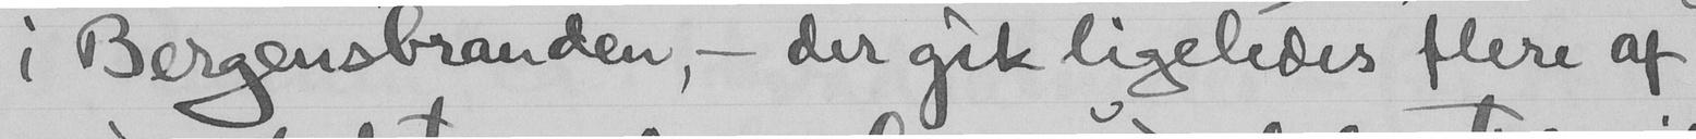i Bergensbranden - der gik ligeledes flere af
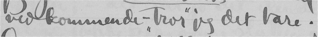vedkommende - "tror" jeg det bare.
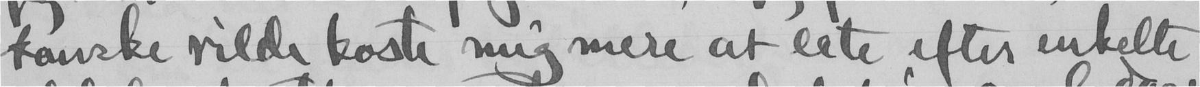kanske vilde koste mig mere at lete efter enkelte
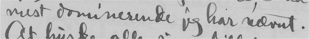mest dominerende jeg har nævnt.
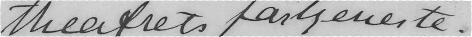theatret fortjeneste.
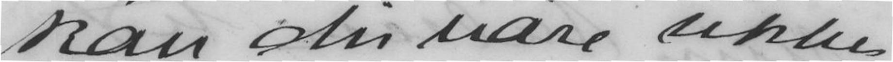kan du være sikker
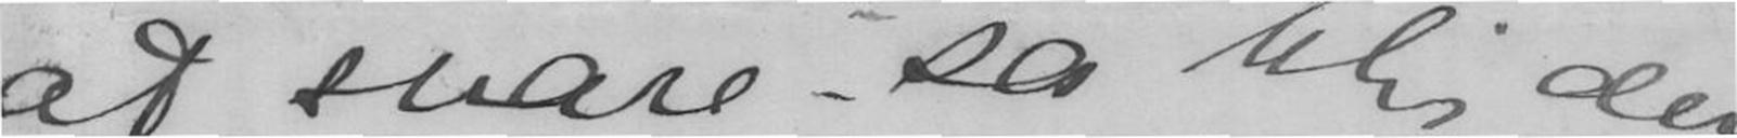at svare - så blir der
